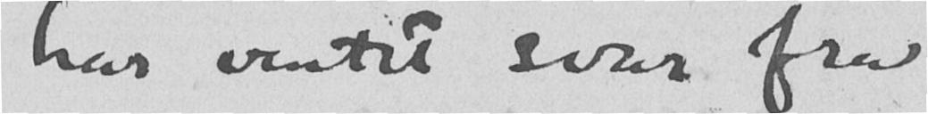har ventet svar fra
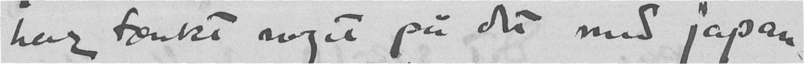har tænkt noget på det med japan
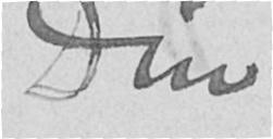Din
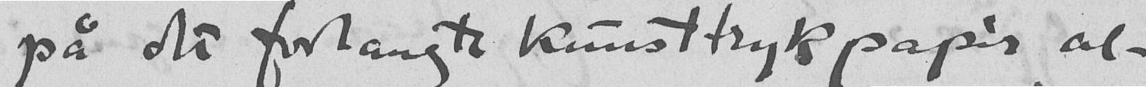på det forlangte kunsttrykpapir al-
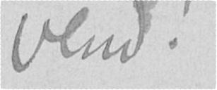vend!
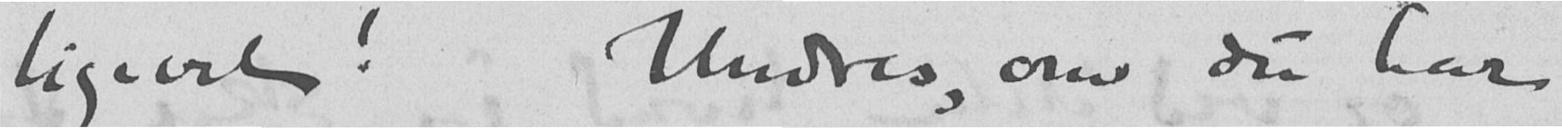ligevel! Undres, om du har
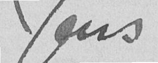Jens
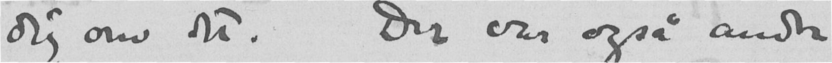dig om det. Der var også andre
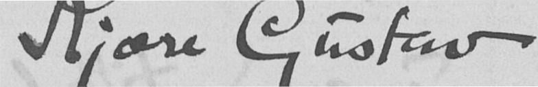Kjære Gustav
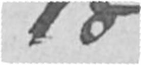18
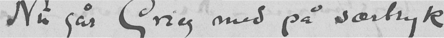Nu går Grieg med på særtryk
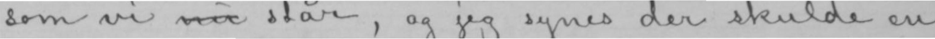som vi nu står, og jeg synes der skulde en
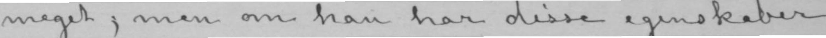meget; men om han har disse egenskaber
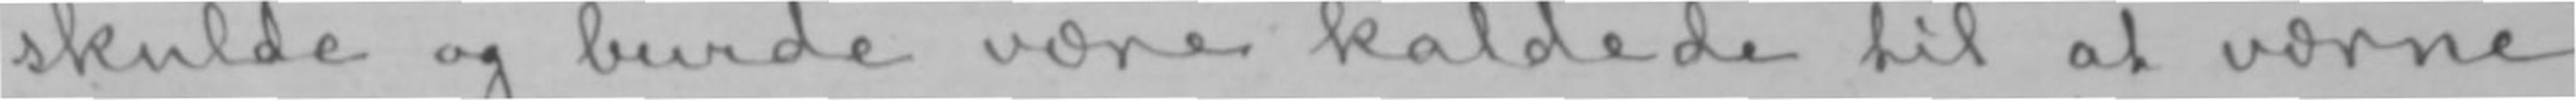skulde og burde være kaldede til at værne
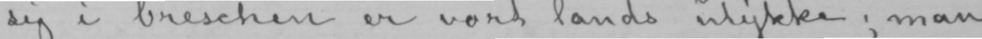sig i breschen er vort lands ulykke; man
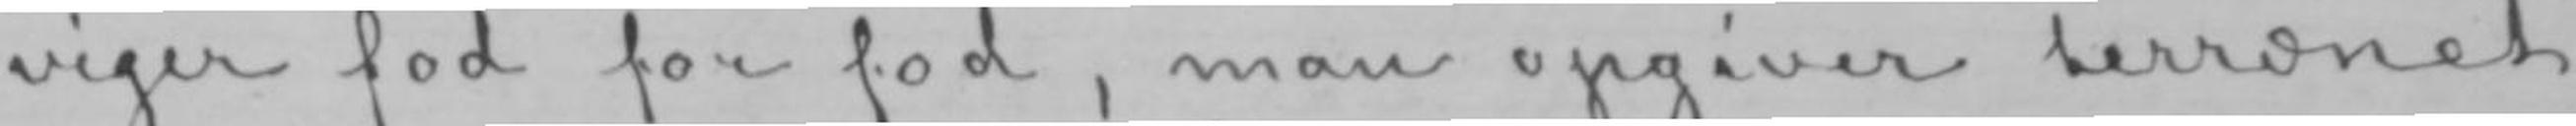viger fod for fod, man opgiver terrænet
